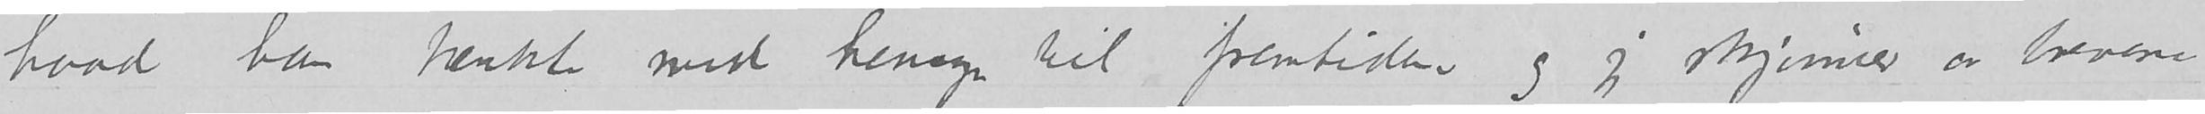hvad han tænkte med hensyn til fremtiden og jeg skjønner av brevene
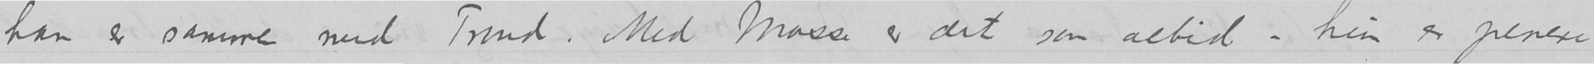han er sammen med Trond.  Med Mosse er det som altid – hun er penere
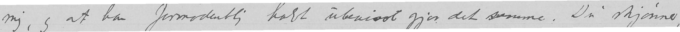mig, og at han formodentlig halvt ubevisst gjør det samme.  Du skjønner,
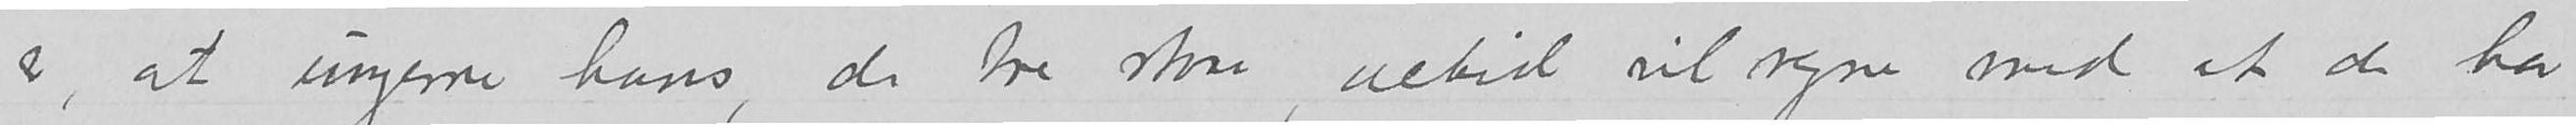er, at ungerne hans, de tre store, altid vil regne med at de har
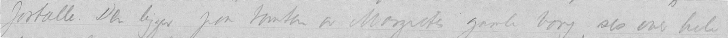fortælle. Den ligger paa tomten av Margretes gamle borg, ses over hele
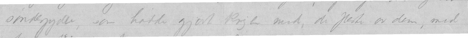sønderjyder, som hadde gjort krigen med, de fleste av dem, med
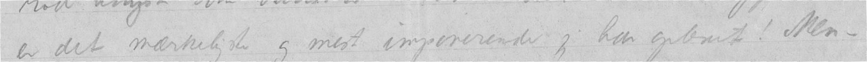er det mærkeligste og mest imponerende jeg har oplevet! Men –
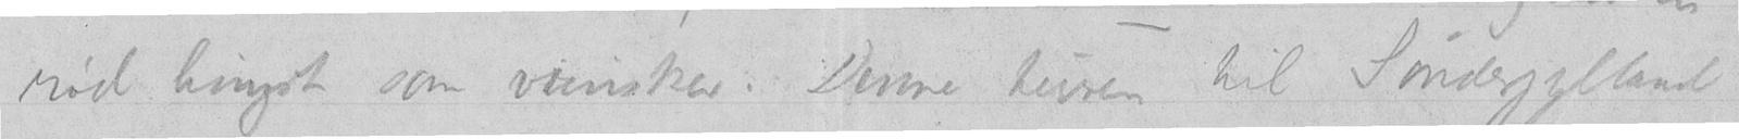rød hingst som vrinsker. Denne turen til Sønderjylland
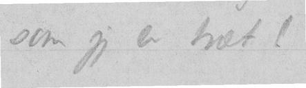som jeg er træt!
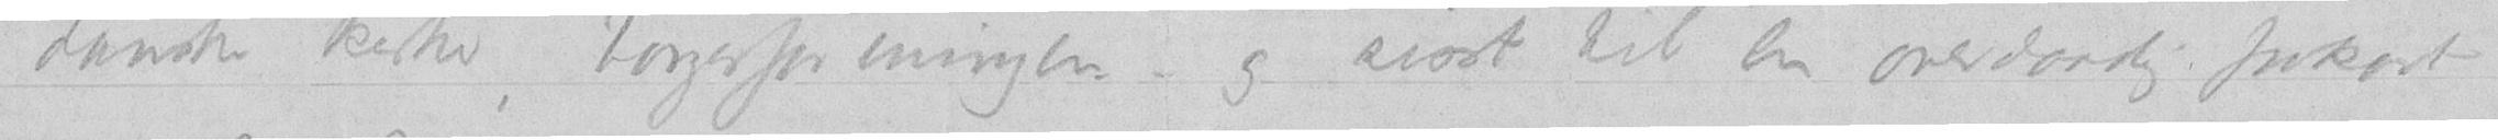danske kirke, borgerforeningen – og sisst til en overdaadig frokost
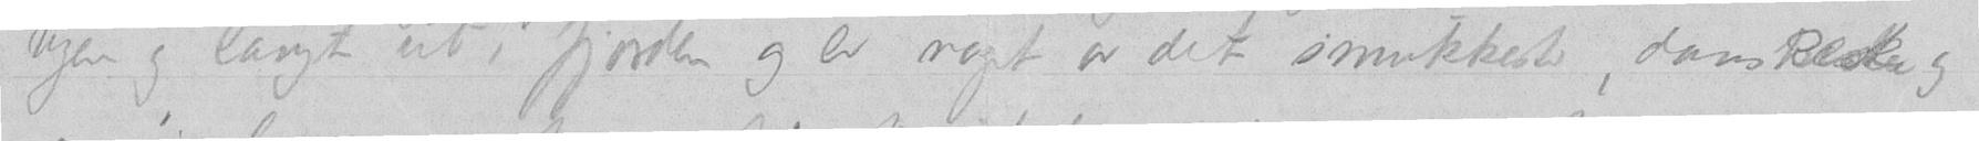byen og langt ut i fjorden og er noget av det smukkeste, danskeste og
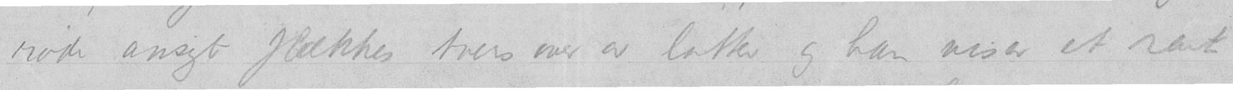røde ansigt flækkes tvers over av latter og han viser et rent
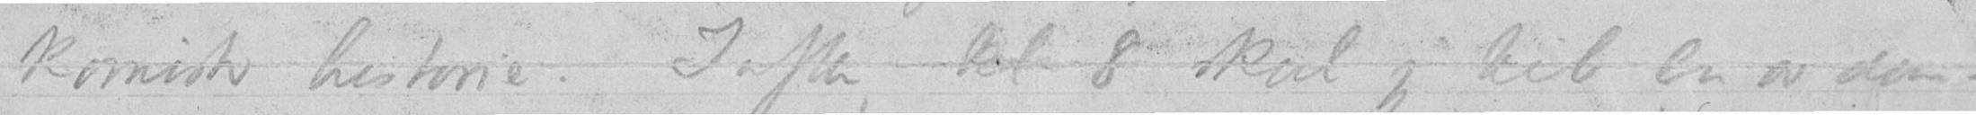komisk historie. Iaften kl. 8 skal jeg til en av dem –
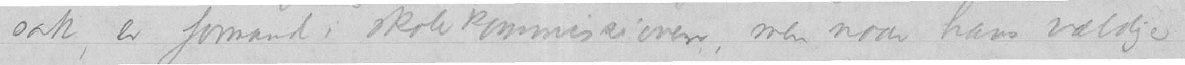sak, er formand i skolekommissionen, men naar hans vældige
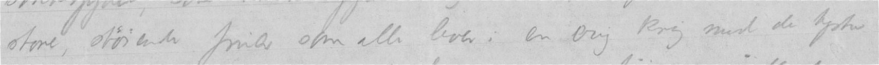store, støiende fruer som alle lever i en ævig krig med de tyske
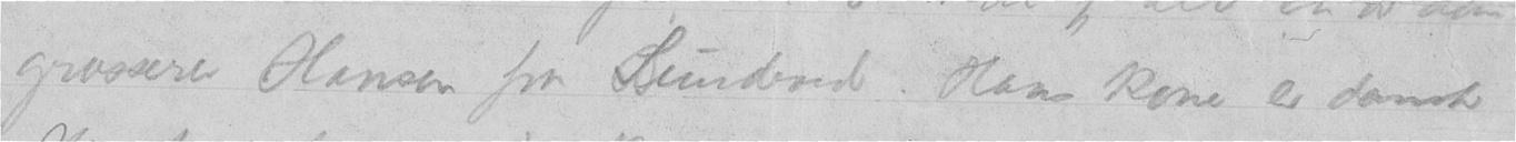grosserer Hansen fra Sundered. Hans kone er dansk
